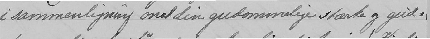i sammenligning med din gudommelige stærke og gud-
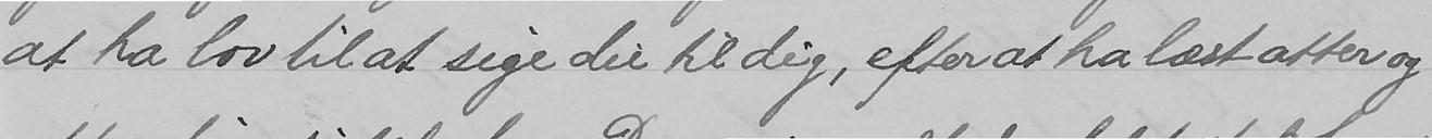at ha lov til at sige du til dig, efter at ha læst atter og
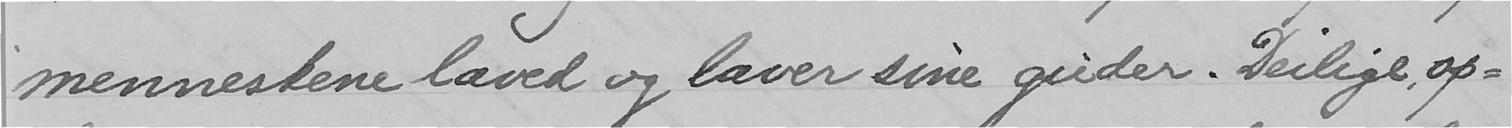mennestene laved og laver sine guder. Deilige op-
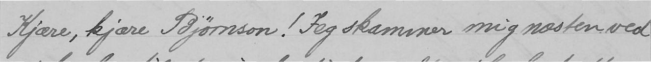Kjære, kjære Bjørnson! Jeg skammer mig næsten ved
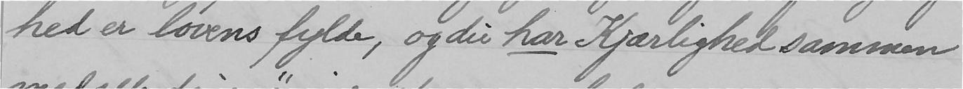hed er lovens fylde, og du har Kjærlighed sammen
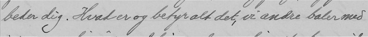beder dig. Hvad er og betyr alt det, vi andre baler med
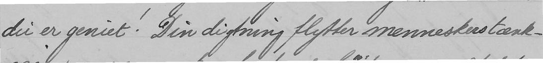du er geniet! Din digtning flytter menneskers tænk-
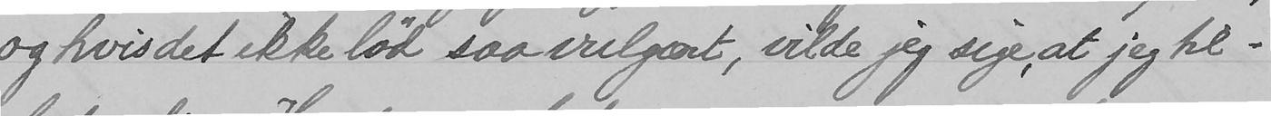og hvis det ikke lød saa vulgært, vilde jeg sige, at jeg til-
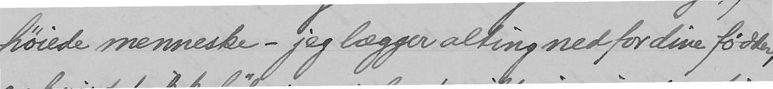høiede menneske - jeg lægger alting ned for dine fødder,
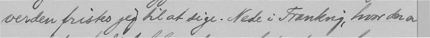verden fristes jeg til at sige. Nede i Frankrig, hvor der er
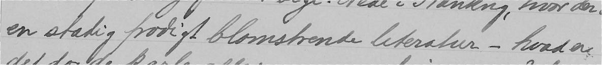en stadig frodigt blomstrende literatur - hvad er
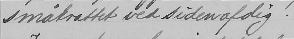småkrattet ved siden af dig!
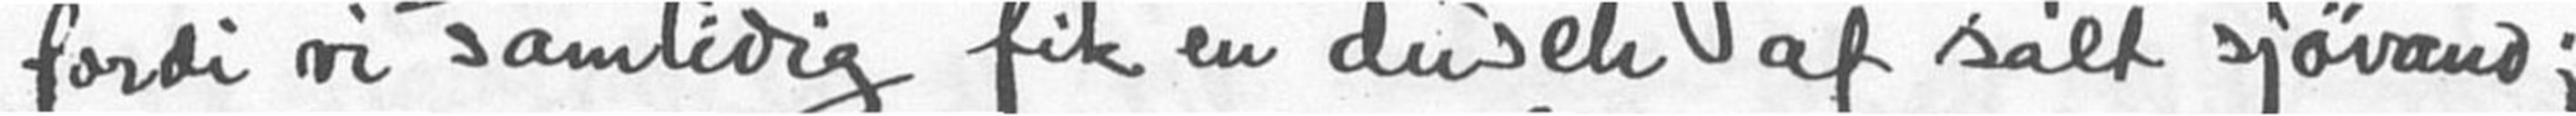fordi vi samtidig fik en dusch af salt sjøvand ;
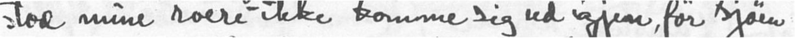stod mine roere - ikke komme sig ud igjen, for sjøen
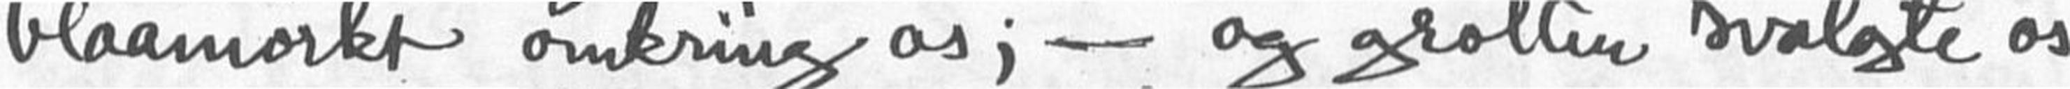blaamørkt omkring os; - og grotten svælgte os
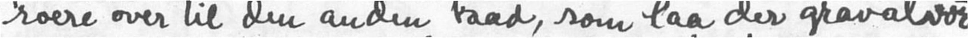roere over til den anden baad, som laa der gravalvor-
